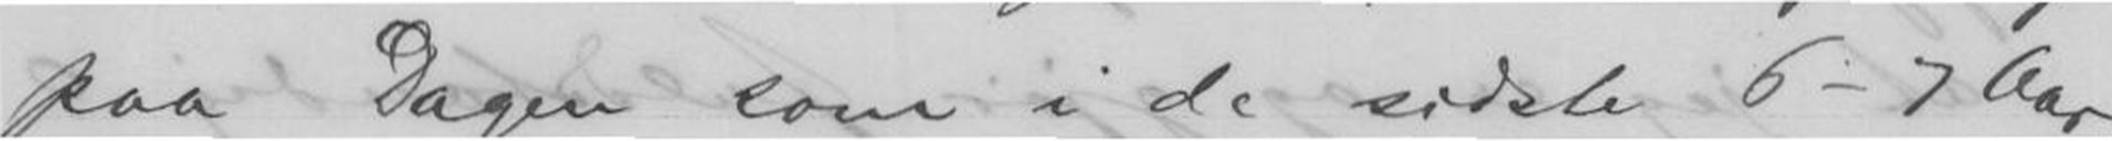paa Dagen som i de sidste 6-7 Aar.
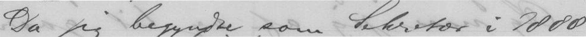Da jeg begyndte som Sekretær i 1888
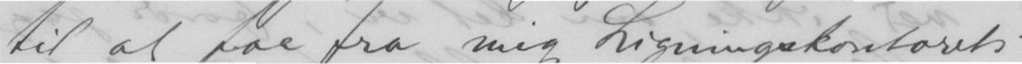til at faa fra mig Ligningskontorets
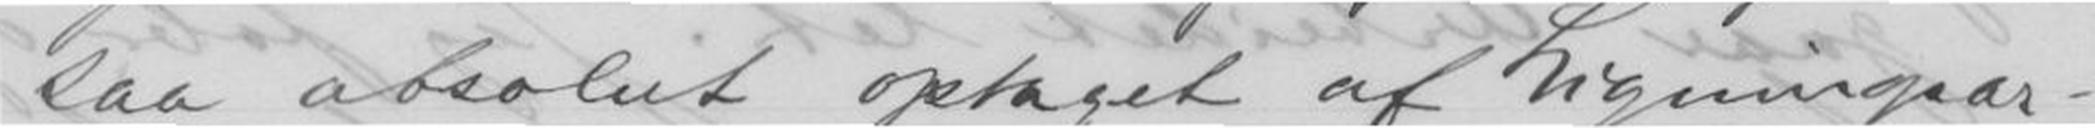saa absolut optaget af Ligningsar-
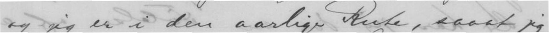og jeg er i den aarlige Rute, saaat jeg
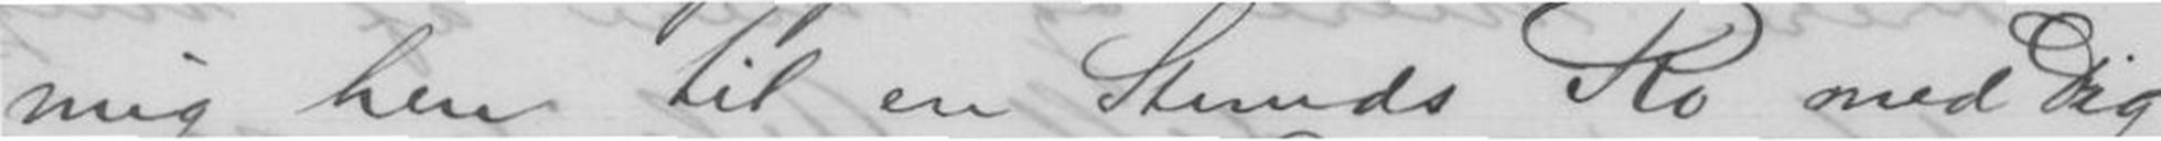mig hen til en Stunds Ro med Dig
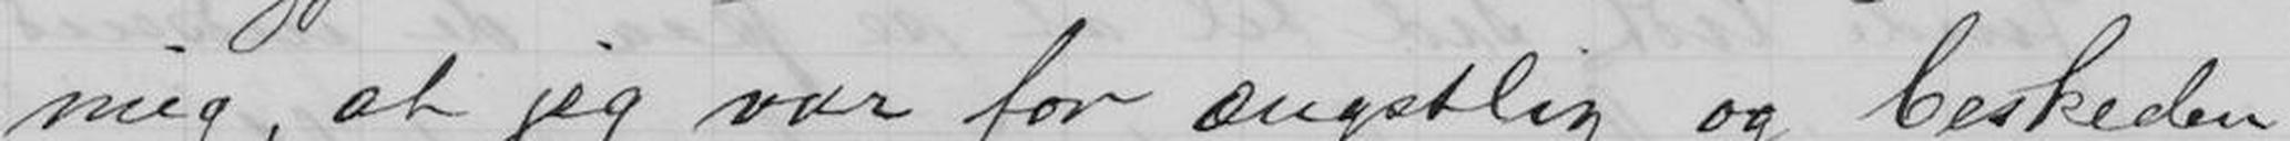mig, at jeg var for ængstlig og beskeden
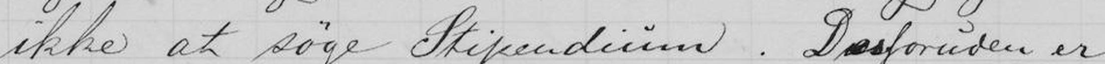ikke at søge Stipendium. Desforuden er
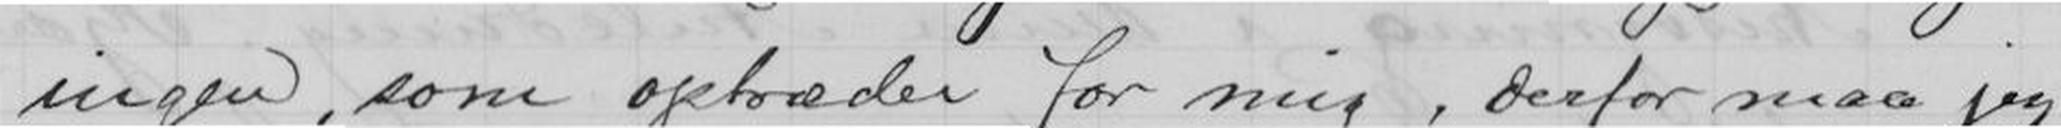ingen, som optræder for mig, derfor maa jeg
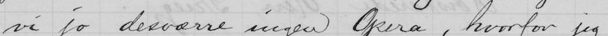vi jo desværre ingen Opera, hvorfor jeg
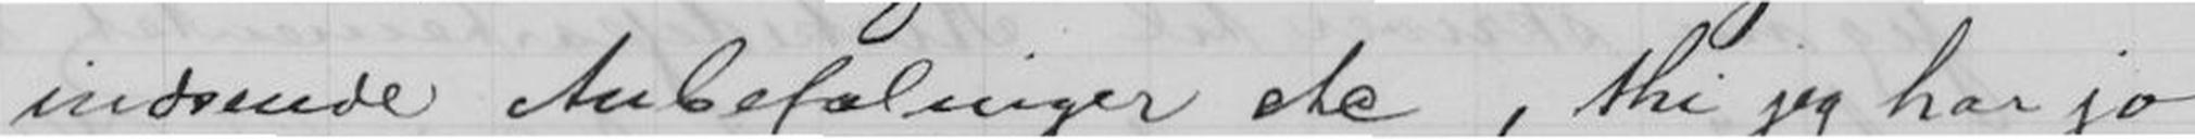indsende Anbefalinger etc, thi jeg har jo
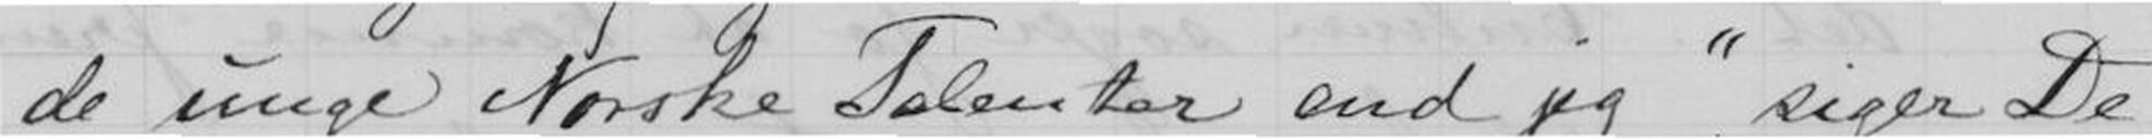de unge Norske Talenter end jeg", siger De
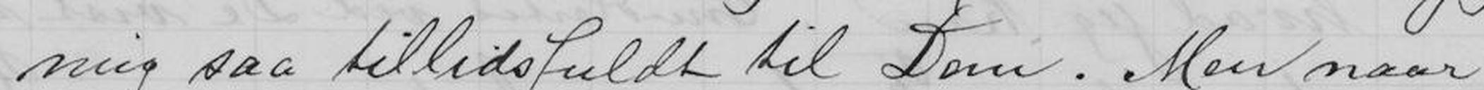mig saa tillidsfuldt til Dem. Men naar
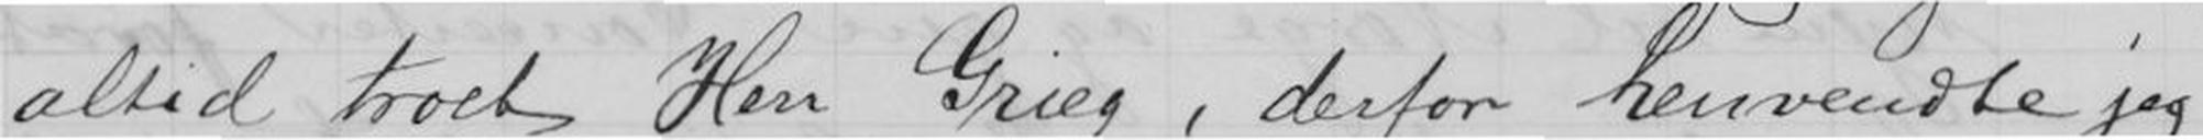altid troet Herr Grieg, derfor henvendte jeg
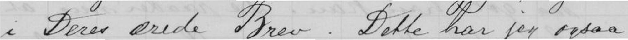i Deres ærede Brev. Dette har jeg ogsaa
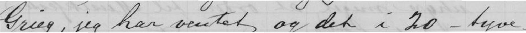Grieg, jeg har ventet og det i 20-tyve-
